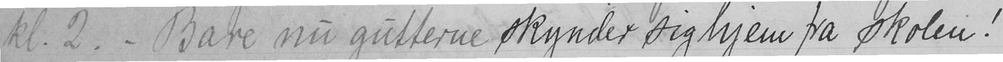kl. 2. - Bare nu gutterne skynder sig hjem fra skolen!
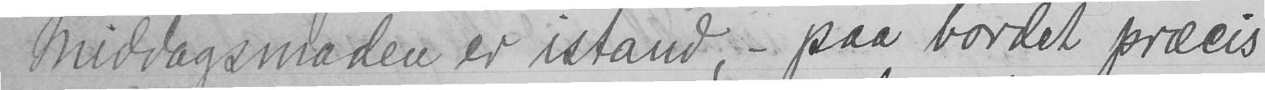Middagsmaden er istand, - paa bordet præcis
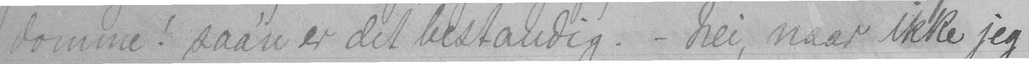domme! saan er det bestandig. - Nei, naar ikke jeg
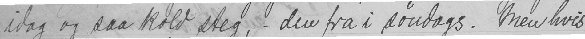idag og saa kold steg, - den fra i søndags. Men hvis
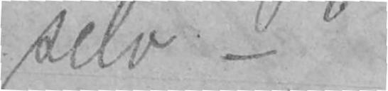selv - "
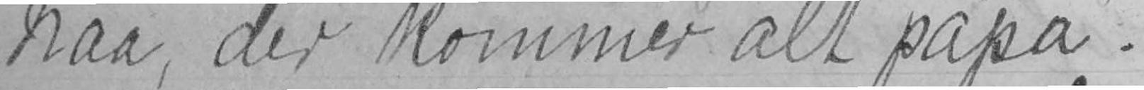naa, der kommer alt papa.
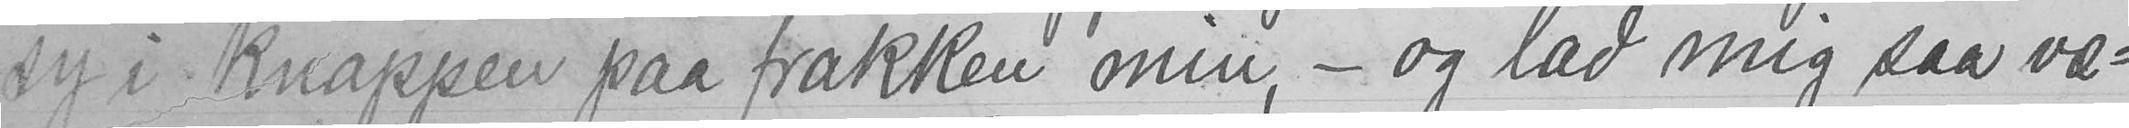sy i knappen paa frakken min, - og lad mig saa væ-
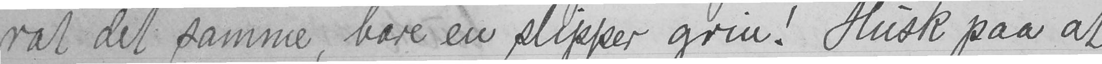rat det samme, bare en slipper grin! Husk paa at
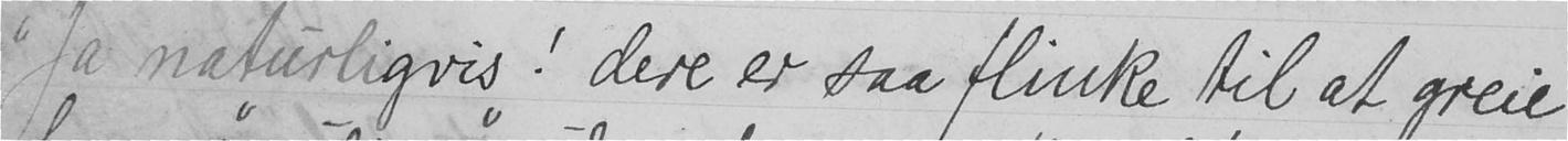"Ja naturligvis! dere er saa flinke til at greie
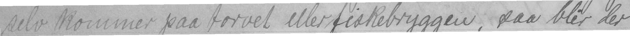selv kommer paa torvet eller fiskebryggen, saa blir der
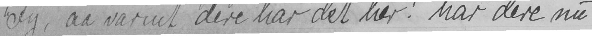"Fy, aa varmt dere har det her! har dere nu
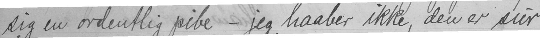sig en ordentlig pibe - jeg haaber ikke, den er sur
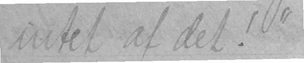intet af det!"
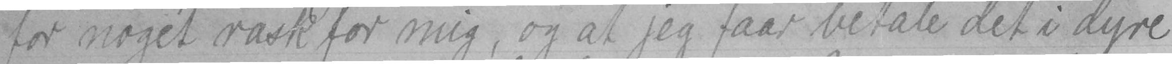for noget rask for mig, og at jeg faar betale det i dyre
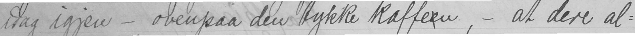idag igjen - ovenpaa den tykke kaffeen, - at dere al-
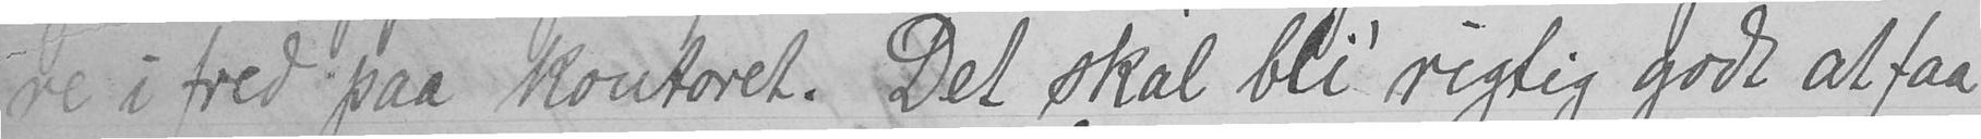re i fred paa kontoret. Det skal bli rigtig godt at faa
